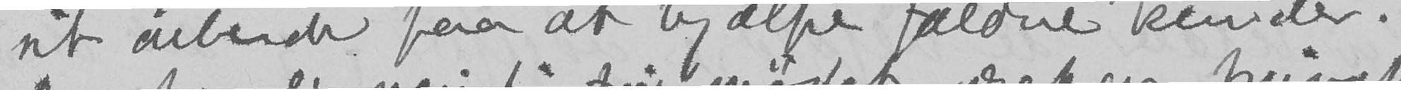sit arbeide paa at hjælpe faldne kvinder.
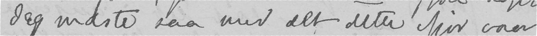Jeg maste saa med alt dette ifjor vaar,
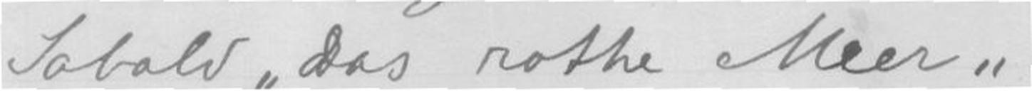Sobald "Das rothe Meer"
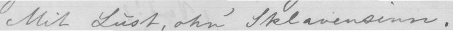Mit Lust, ohn' Sklavensinn.
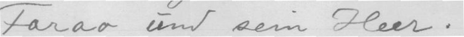Farao und sein Heer.
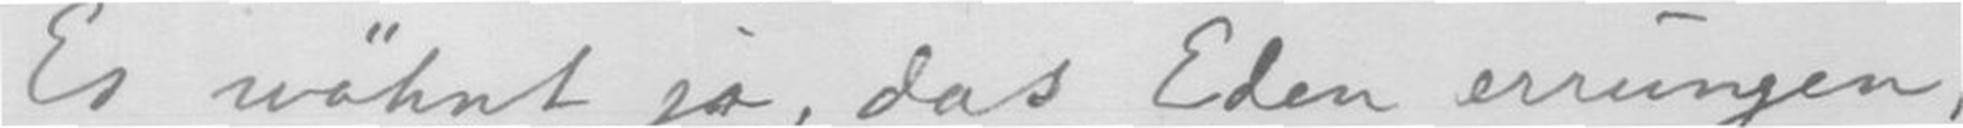Es wöhnt ja, das Eden errungen,
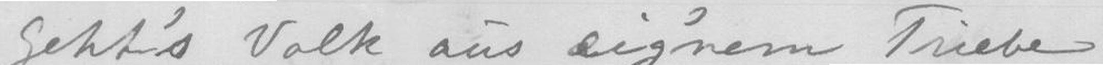Geht's Volk aus sig'nem Triebe
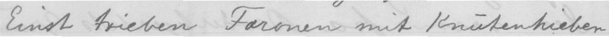Einst trieben Faronen mit Knutenhieben
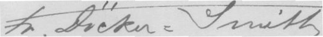Fr. Döcker=Smith.
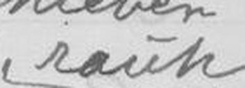rauh
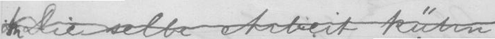Die selbe Arbeit kühn
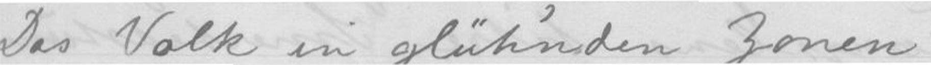Das Volk in glüh'nden Zonen
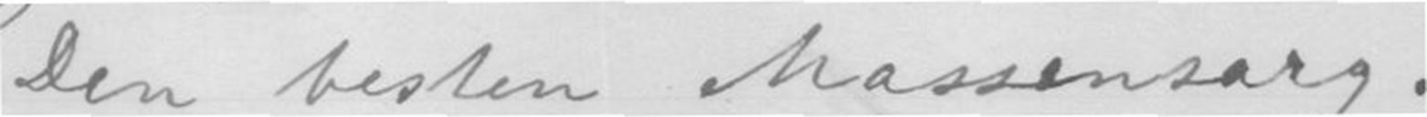Den besten Massensarg.
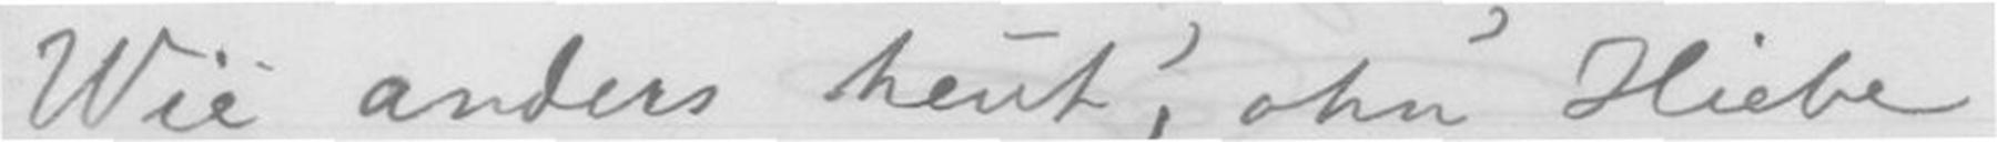Wie anders heut', ohn' Hiebe
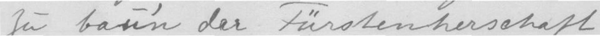Zu bau'n der Fürstenherschaft
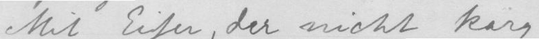Mit Eifer, der nicht kärg.
